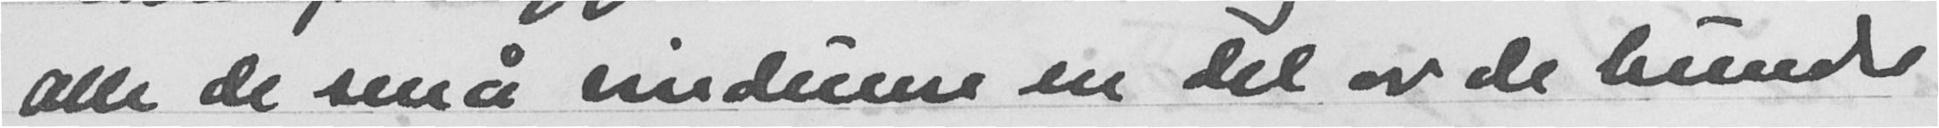alle de små vinduene en del av de hundre
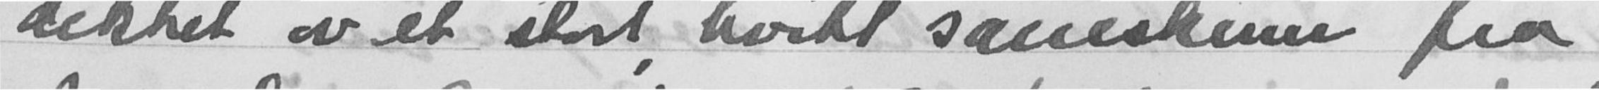dekket av et stort hvitt saueskinn fra
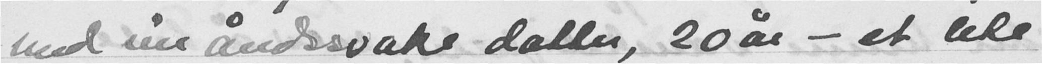med sin åndssvake datter, 20 år - et lite
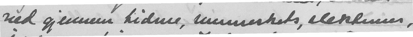ned gjennem tidene, menneskets, slekternes,
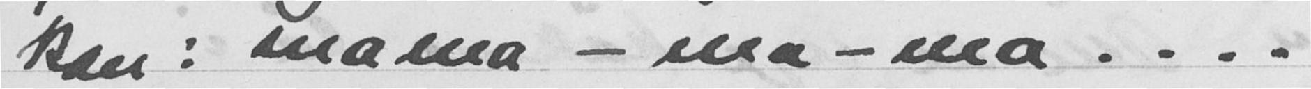kan: mama - ma-ma....
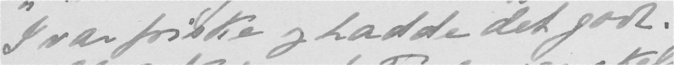I var friske og hadde det godt.
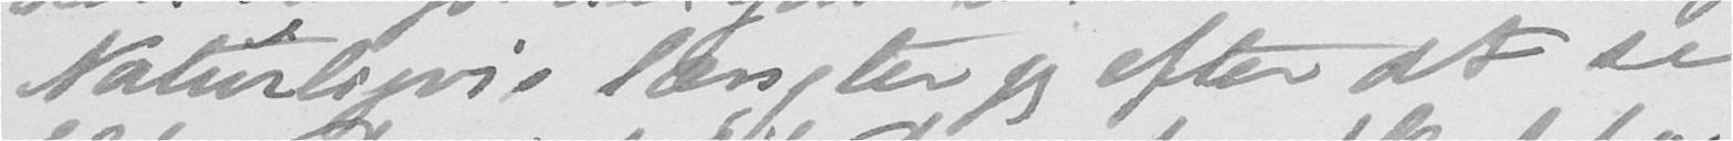Naturligvis længter jeg efter at se
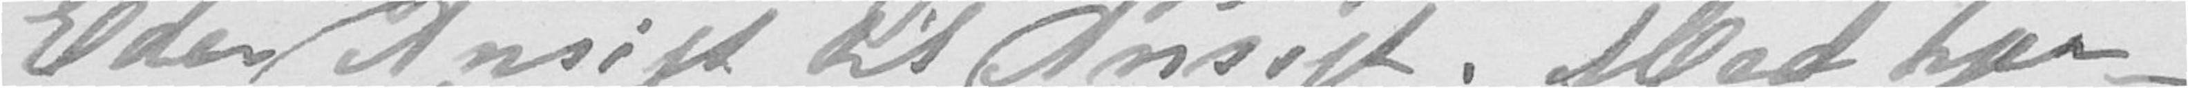Eder Ansigt til Ansigt. Med hjer-
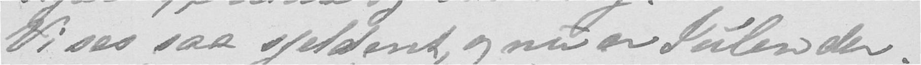Vi ses saa sieldent, og nu er Julen der.
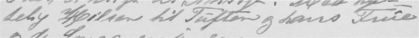telig Hilsen til Tuften og hans Frue
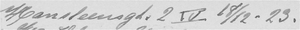Hansteensgt. 2 IV 18/12-23.
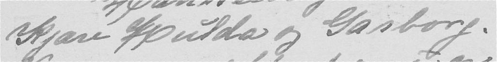Kjære Hulda og Garborg
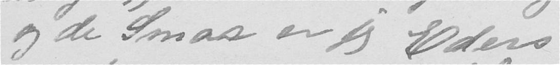og de Smaa er jeg Eders
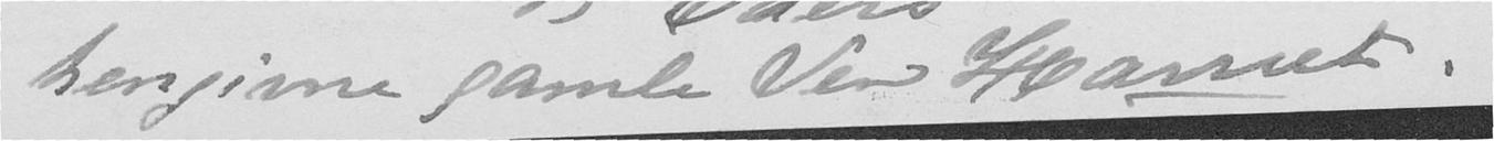hengivne gamle Ven Harriet
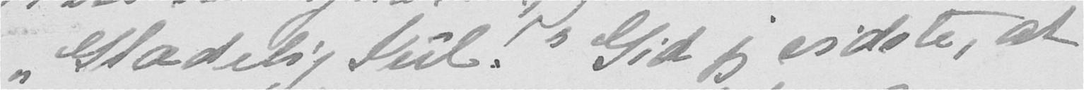"Glædelig Jul!" Gid jeg vidste, at
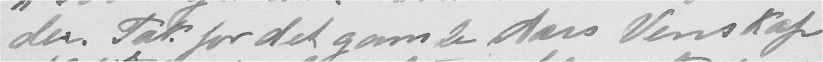der. Tak for det gamle Aars Venskap
